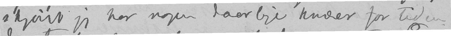skjønt jeg har nogen daarlige knæer for tiden.
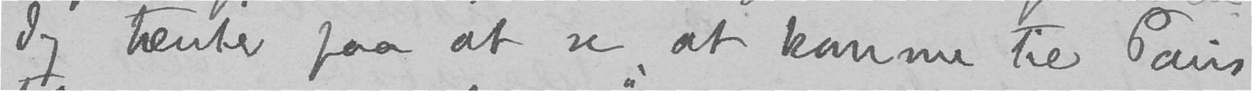Jeg tænker paa at se at komme til Paris
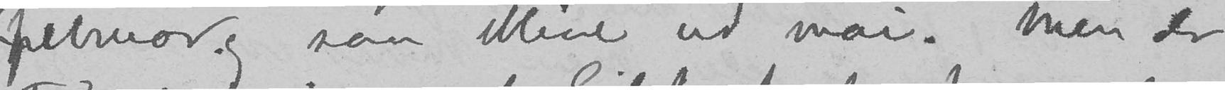februar og saa blive ud mai. Men der
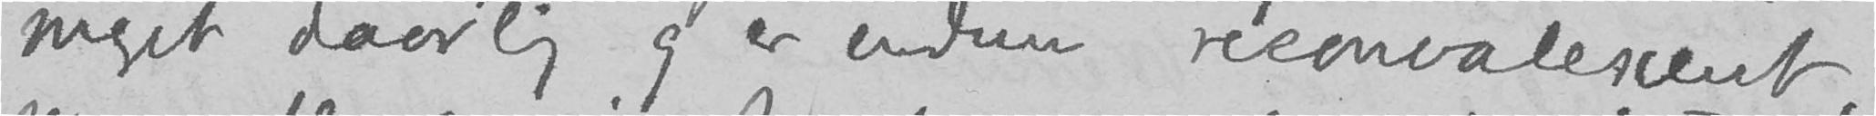meget daarlig og er endnu reconvalescent,
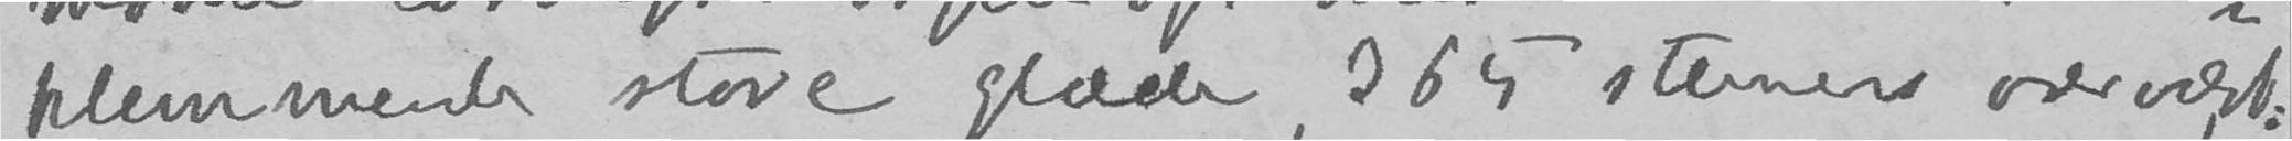klemmende store glæde, 265 stemers overvægt.
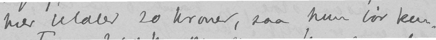hver betaler 20 kroner, saa hun bør kun-
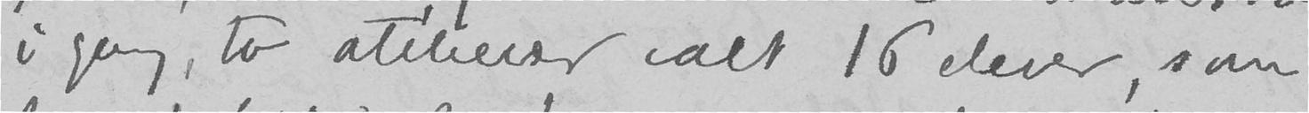i gang, to atelierer ialt 16 elever, som
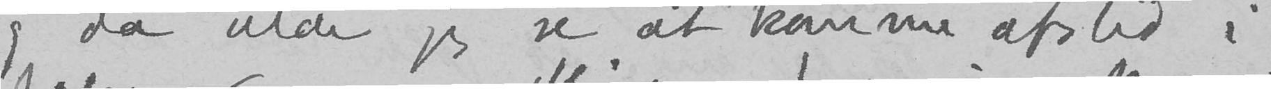og da vilde jeg se at komme afsted i
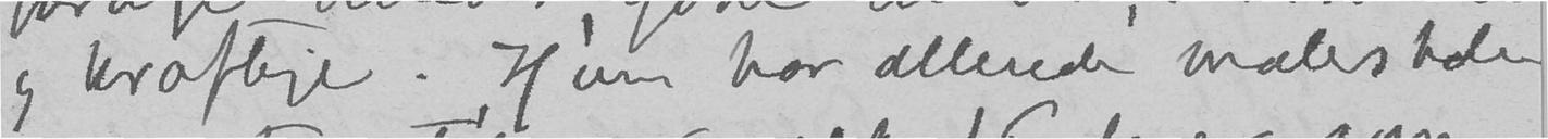og kraftige. Hun har allerede maleskole
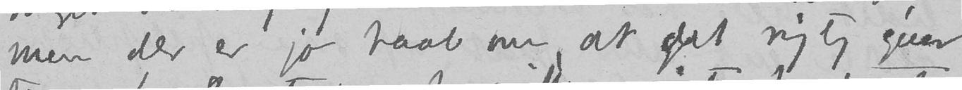men der er jo haab om at det rigtig gaar
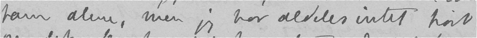ham alene, men jeg har aldeles intet hørt
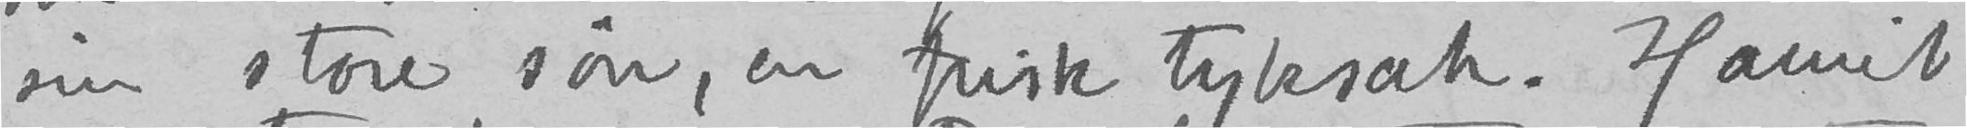sin store søn, en frisk tyksak. Harriet
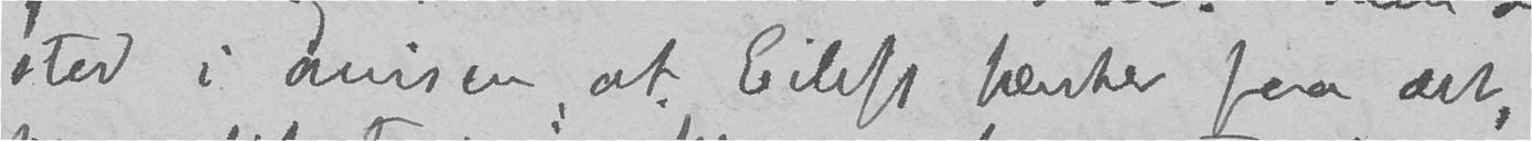stod i avisen at Eilifs tænker paa det,
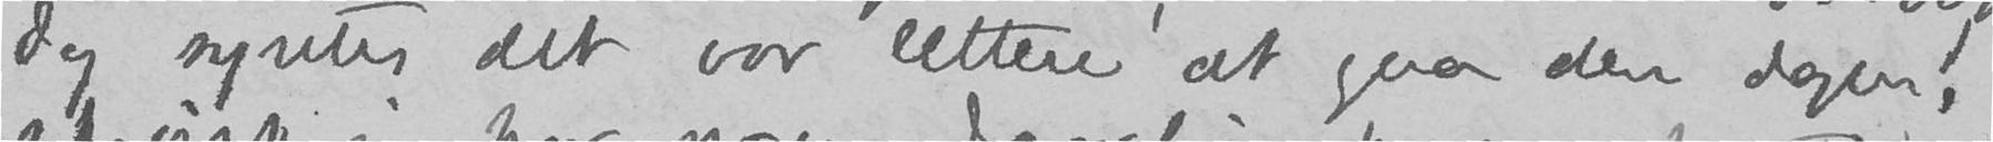Jeg syntes det var lettere at gaa den dagen,
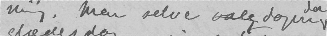ning. Men selve valgdagen
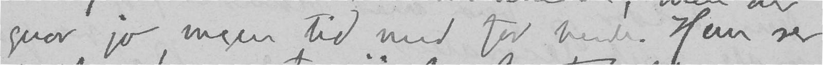gaar jo megen tid med for hende. Hun ser
